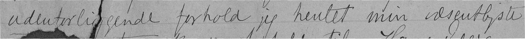udenforliggende forhold jeg hentet min væsentligste
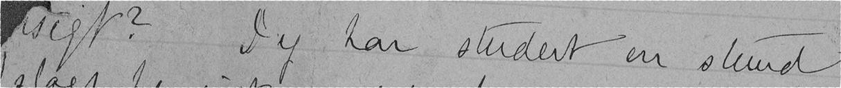sigt? Jeg har studert en stund
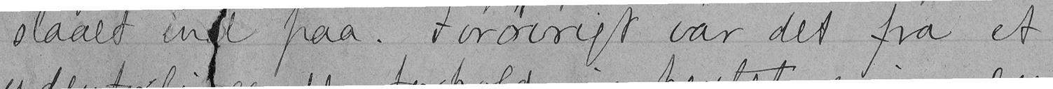slaaet ind paa. Forøvrigt var det fra et
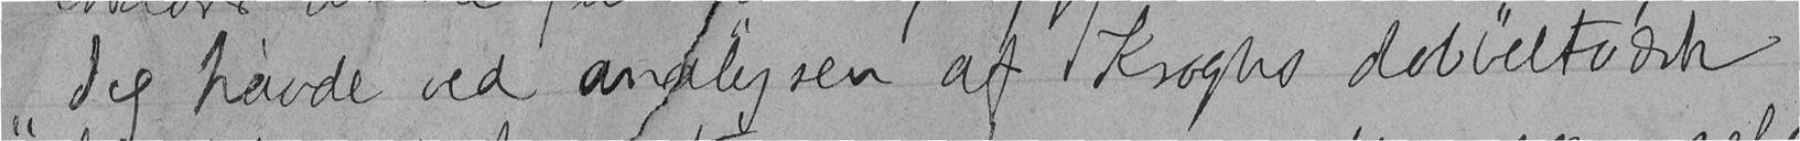"Jeg havde ved analysen af Kroghs dobbeltværk
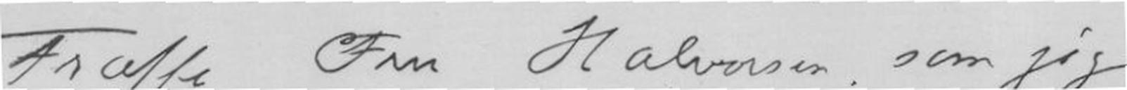Træffe Fru Halvorsen som jeg
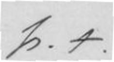p. t.
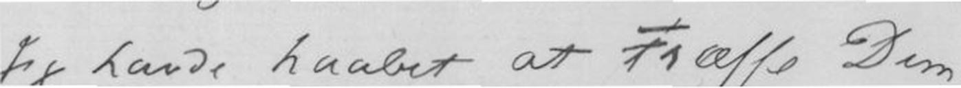Jeg havde haabet at Træffe Dem
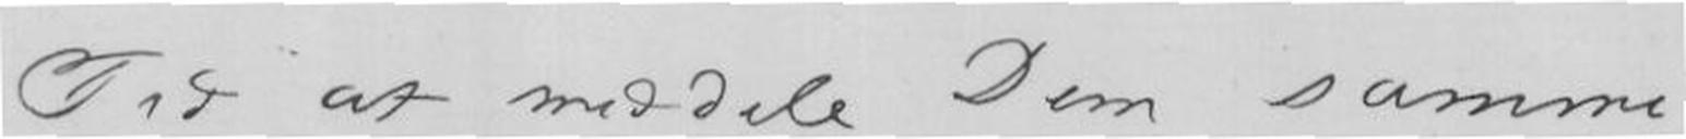Tid at meddele Dem sammen
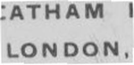LONDON
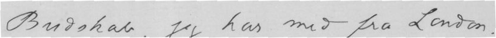Budskab, jeg har med fra London.
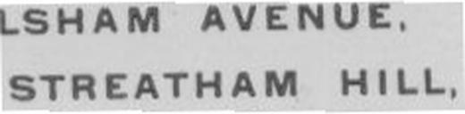STREATHAM HILL.
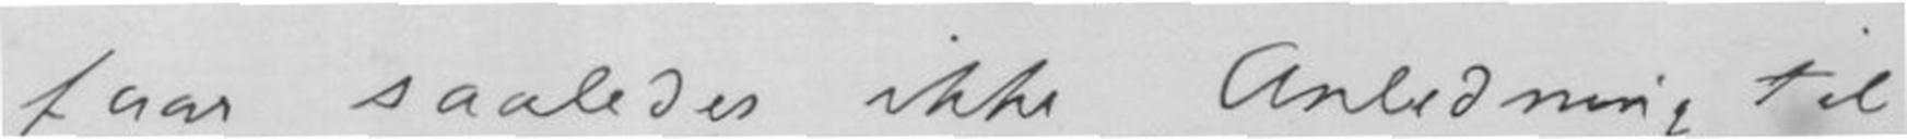faar saaledes ikke Anledning til
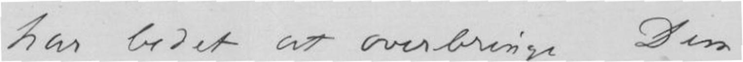har bedet at overbringe Dem
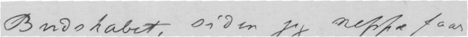Budskabet, siden jeg neppe faar
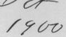1900
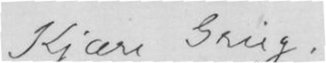Kjære Grieg.
